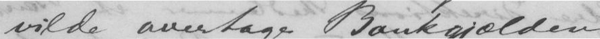vilde overtage Bankgjælden
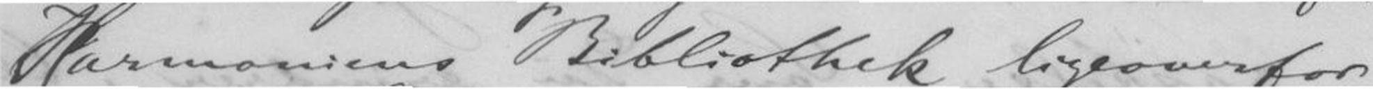Harmoniens Bibliothek ligeoverfor
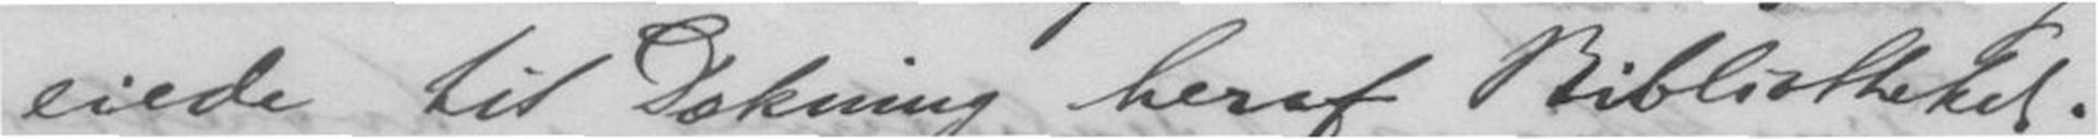eiede til Dækning heraf Bibliotheket.
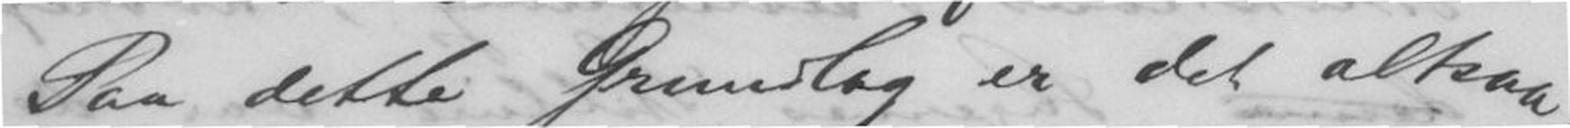Paa dette Grundlag er det altsaa,
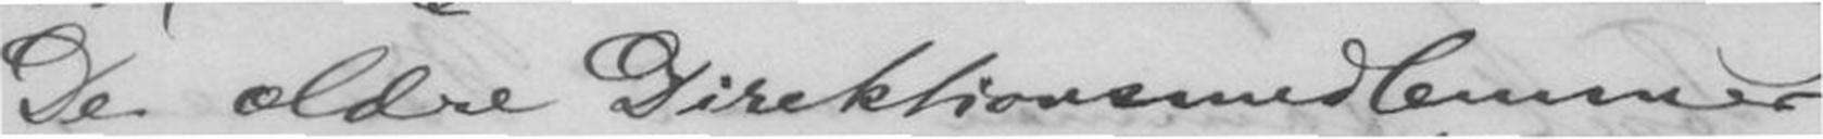De ældre Direktionsmedlemmer
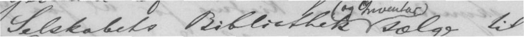Selskabets Bibliothek sælge til
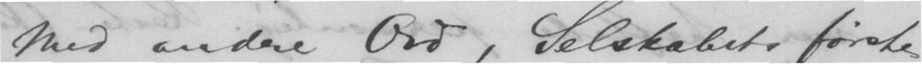Med andre Ord, Selskbets første
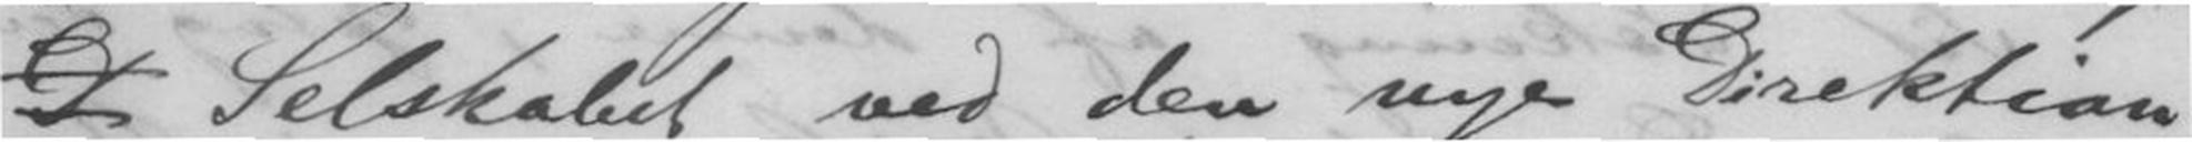D Selskabet ved den nye Direktion
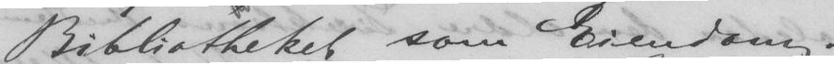Bibliotheket som Eiendom.
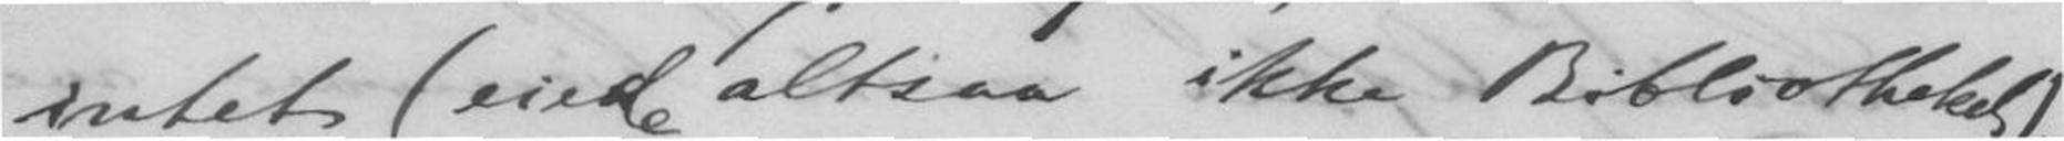intet (eiede altsaa ikke Bibliotheket).
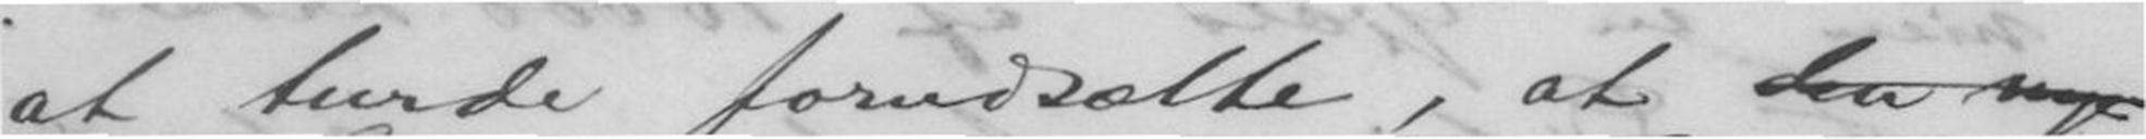at turde forudsætte, at den nye
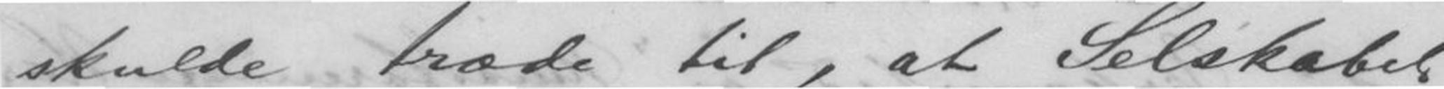skulde træde til, at Selskabet
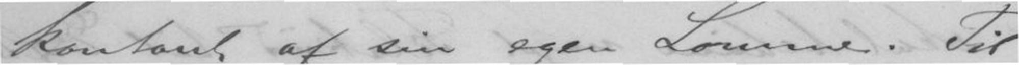kontant af sin egen Lomme. Til
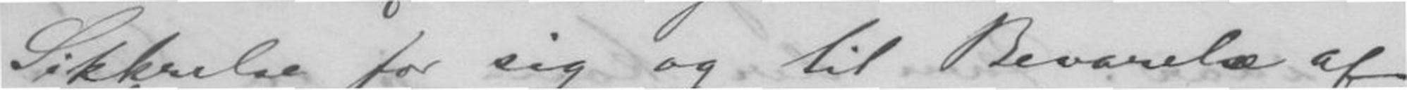Sikkrelse for sig og til Bevarelse af
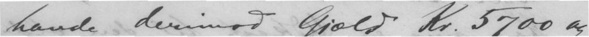havde derimod Gjæld Kr. 5700 og
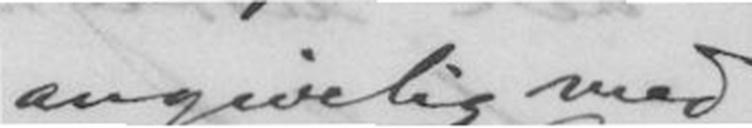rektionen s Medlemmer, angivelig med
